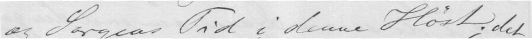og Sorgens Tid i denne Høst; det
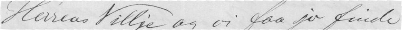Herrens Villje og vi faa jo finde
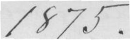1875 Kjære Ven Edvard.
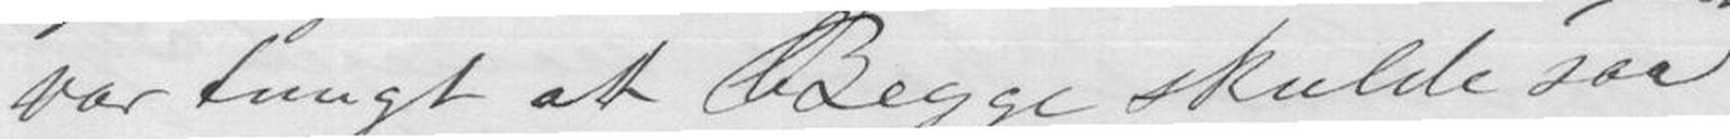var tungt at Begge skulde saa
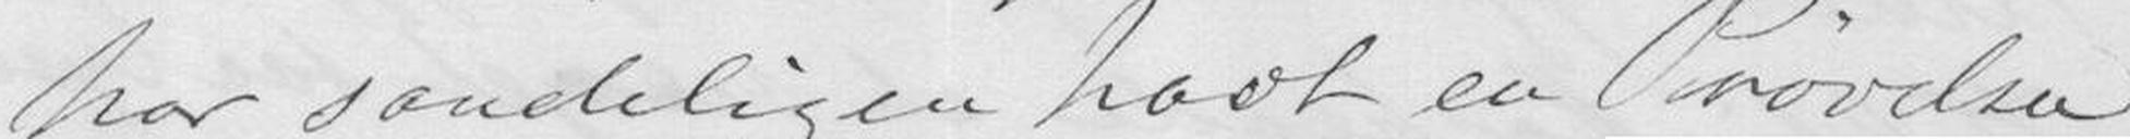har sandeligen havt en Prøvelse
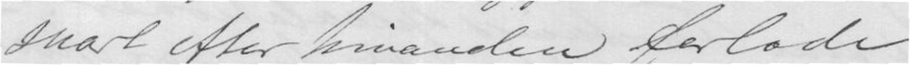snart efter hinanden forlade
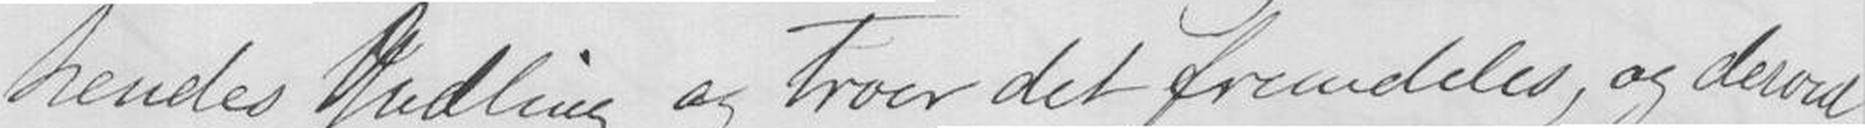hendes Gudling og troer det fremdeles, og derved
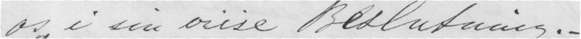os i sin viise Beslutning. -
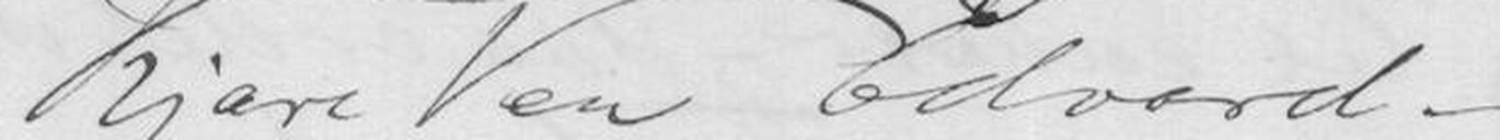Du og Kone Dine kjære Sødskende og
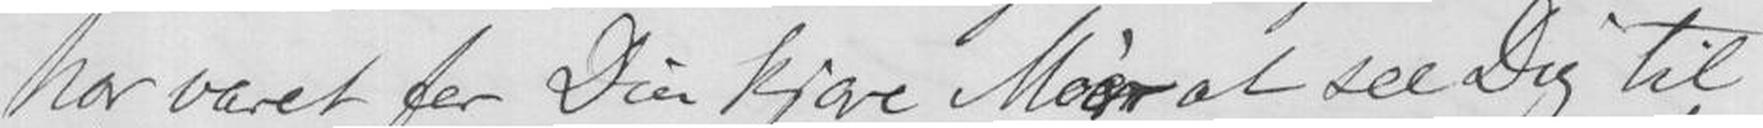har varet for Din kjære Moer at see Dig til
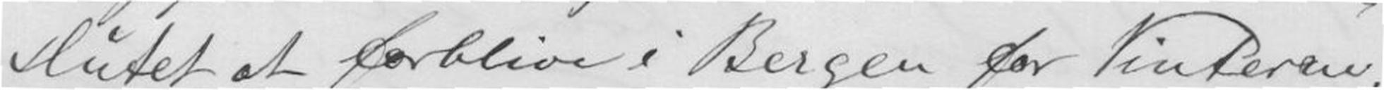slutet at forblive i Bergen for Vinteren,
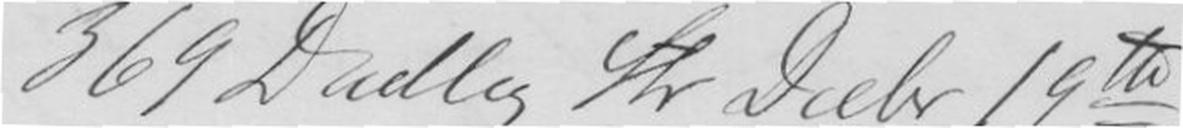369 Dadley Str Decbr 19th
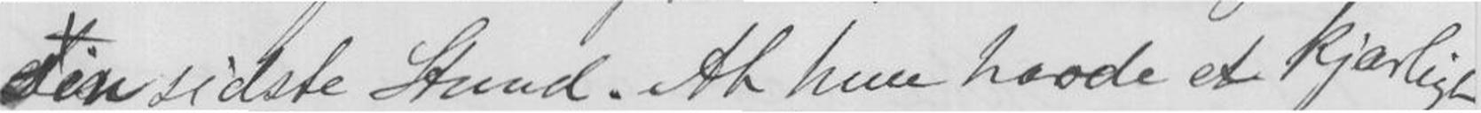den sidste Stund. At hun havde et kjærligt
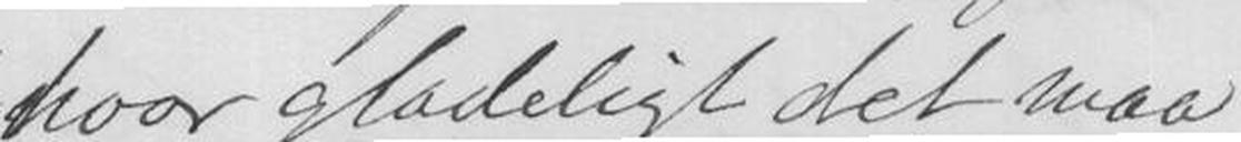hvor glædeligt det maa
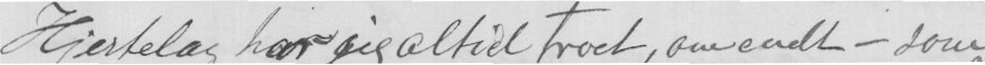Hjertelag har jeg altid troet, om endt - som
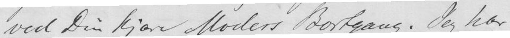ved Din kjære Moders Bortgang. Jeg har
